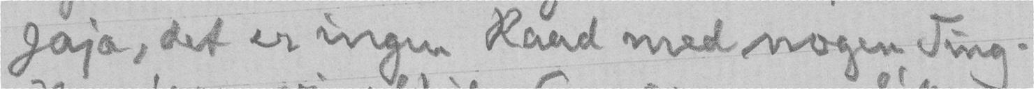Jaja, det er ingen Raad med nogen Ting.
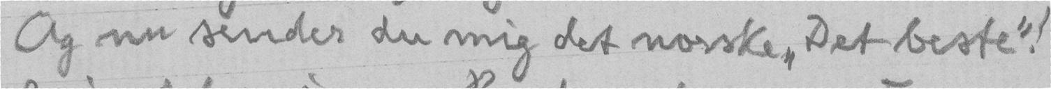Og nu sender du mig det norske "Det beste"!
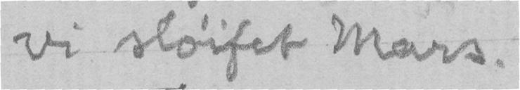vi sløyfet Mars.
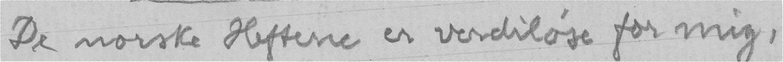De norske Heftene er verdiløse for mig,
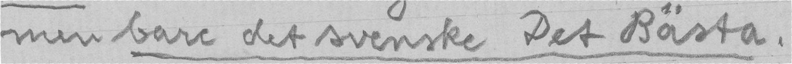men bare det svenske Det Bästa.
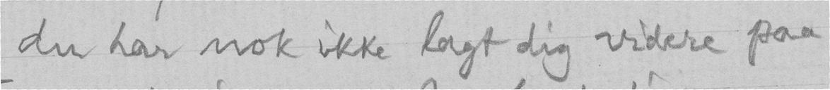du har nok ikke lagt dig videre paa
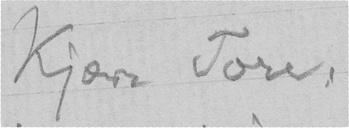Kjære Tore,
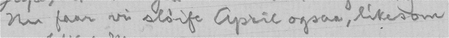Nu faar vi sløyfe April ogsaa, likesom
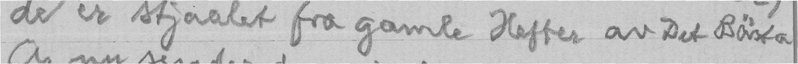de er stjaalet fra gamle Hefter av Det Bästa
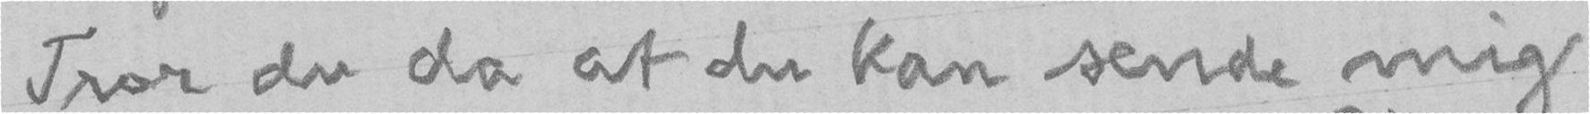Tror du da at du kan sende mig
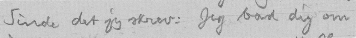Sinde det jeg skrev: Jeg bad dig om
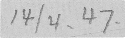14/4. 47.
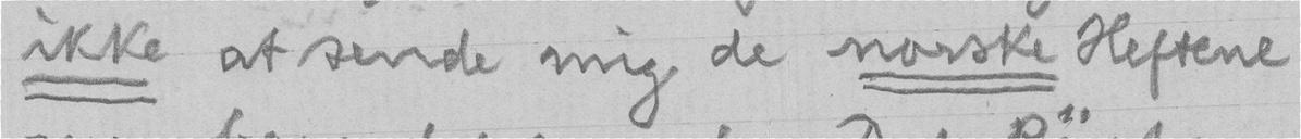ikke at sende mig de norske Heftene
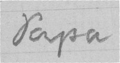Papa
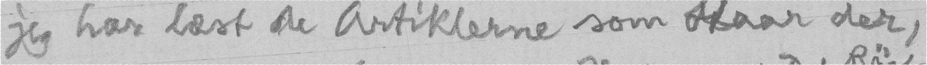jeg har læst de Artiklerne som staar der,
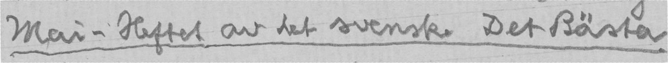Mai-Heftet av det svenske Det Bästa
